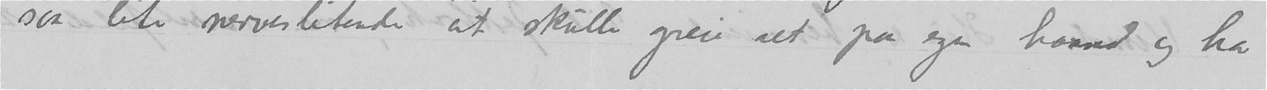saa lite nerveslitende at skulle greie alt paa egen haand og ha
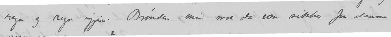regn og regn igjen.  Brønden min maa da være sikker for denne
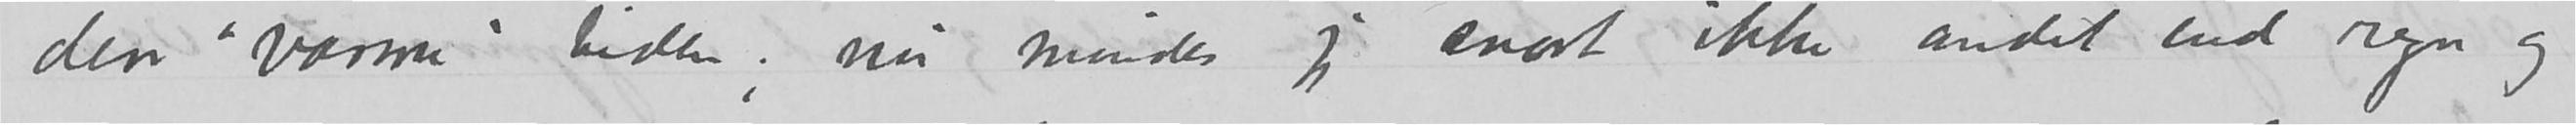den «varme» tiden; nu mindes jeg snart ikke andet end regn og
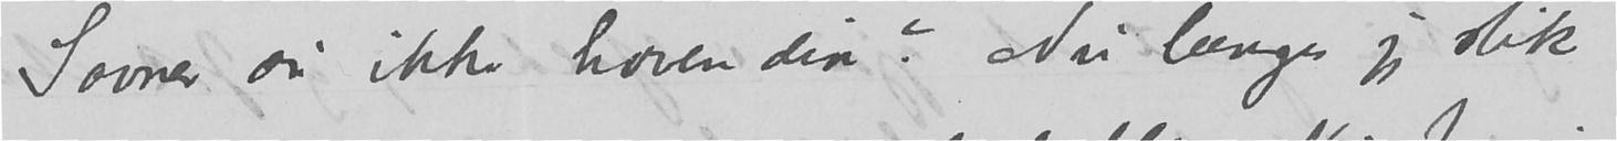Savner du ikke haven din?  Nu længes jeg slik
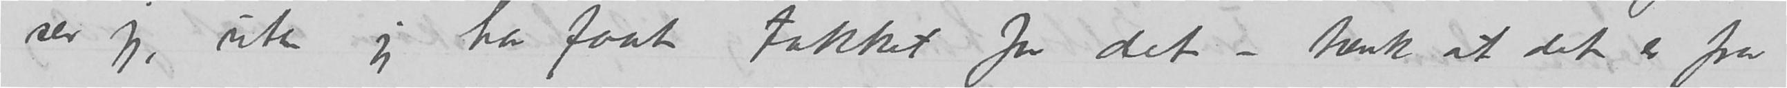ser jeg, uten jeg har faat takket for det -– tænk at det er fra
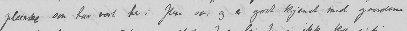pleierske som har vært her i flere aar, og er godt kjendt med gaardene
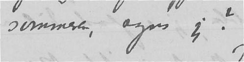sommeren, synes jeg?
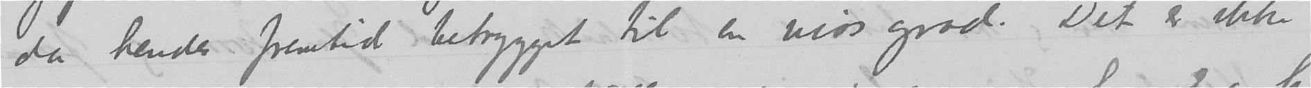da hendes fremtid betrygget til en viss grad.  Det er ikke
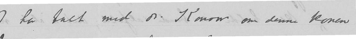Jeg har talt med dr. Konow om denne konen
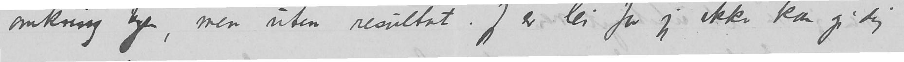omkring byen, men uten resultat.  Jeg er lei for jeg ikke kan gi dig
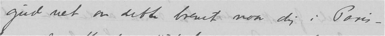gud vet om dette brevet naar dig i Paris –
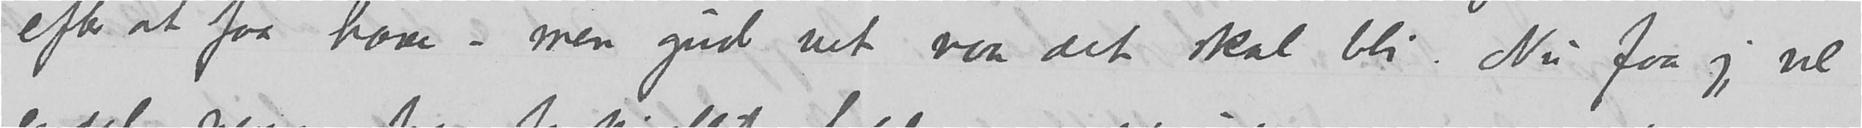efter at faa have – men gud vet naar det skal bli. Nu faar jeg vel
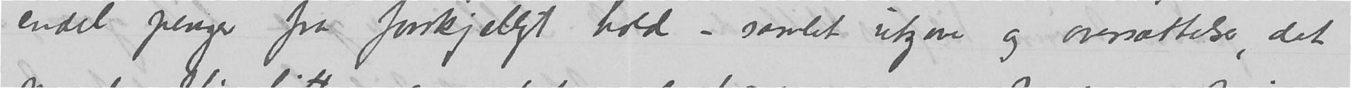en del penger fra forskjeligt hold – samlet utgave og oversættelser, det
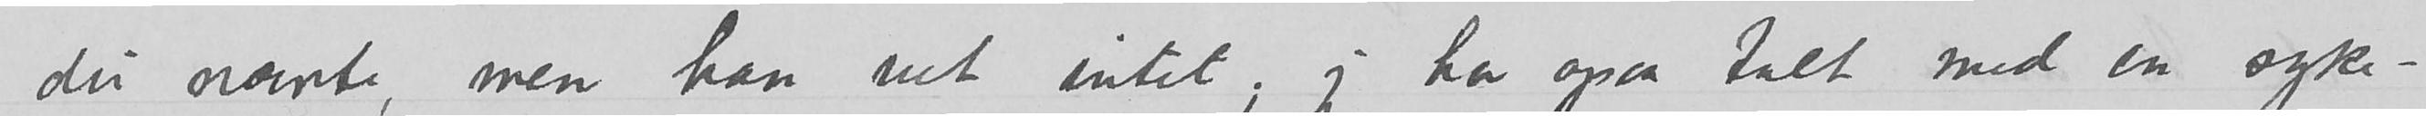du nævnte, men han vet intet; jeg har ogsaa talt med en syke-
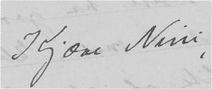Kjære Nini,
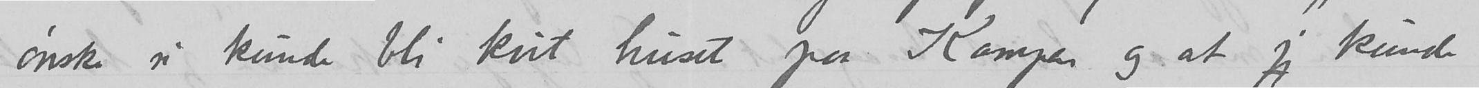ønske vi kunde bli kvit huset paa Kampen og at jeg kunde
